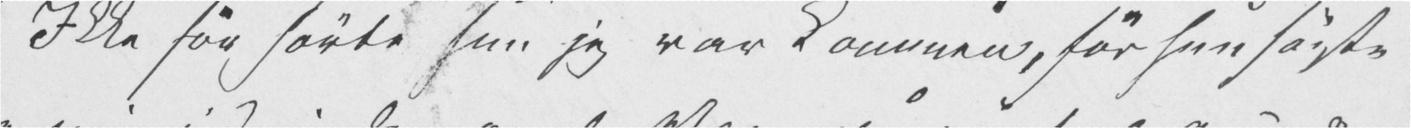Ikke før hørte hun jeg var kommen, før hun søgte
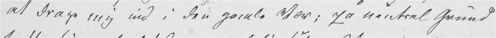at drage mig ind i den gamle Væv, på neutral Grund
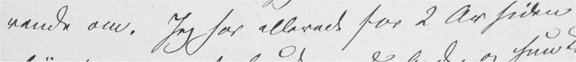vende om. Jeg har allerede for 2 År siden
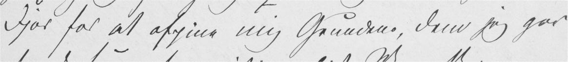Fjor for at afpine mig Grundene, dem jeg gav
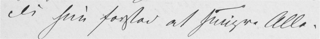di hun forstod at smigre Alle.
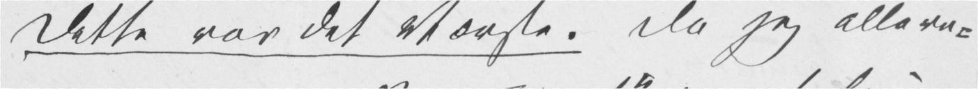Dette var det Værste. Da jeg allere-
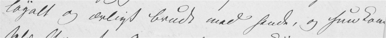loyalt og ærligt brudt med hende, og hun kom
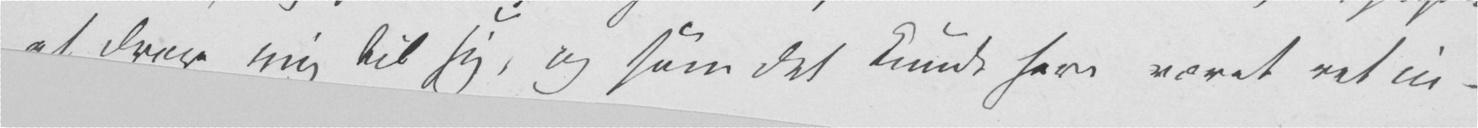at drage mig til sig, og som det kunde have været ret in-
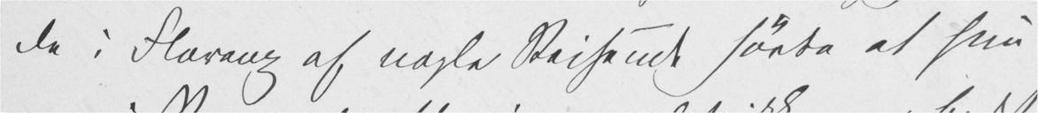de i Florenz af nogle Reisende hørte at hun
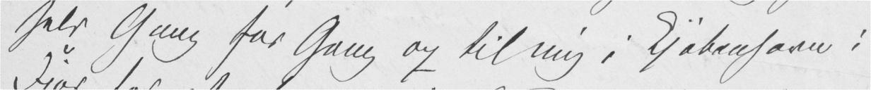selv Gang for Gang op til mig i Kjøbenhavn i
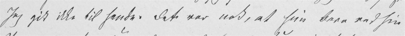Jeg gik ikke til hende. Det var nok, at hun bare ved sin
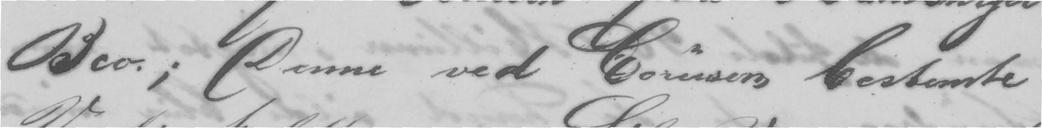Bev.; Denne ved Coursens bestemte
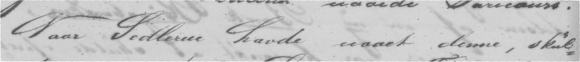Naar Sedlerne havde naaet denne, skul-
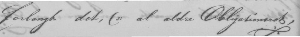forlangt det, (ɔ: al ældre Obligationsræt,
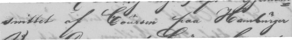snittet af Coursen paa Hamburger
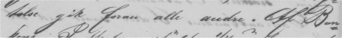telse gik foran alle andre. Af Ban-
